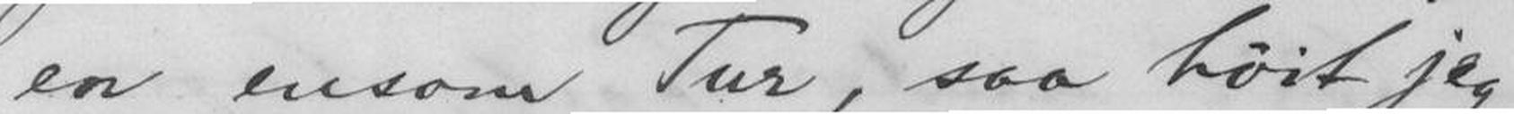en ensom Tur, saa høit jeg
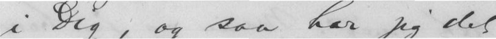i Dig, og saa har jeg det
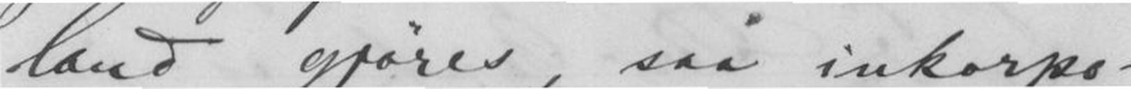land gjøres, saa inkorpo-
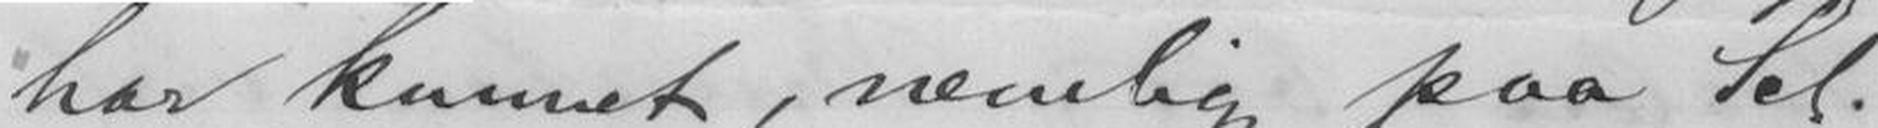har kunnet, nemlig paa Sct.
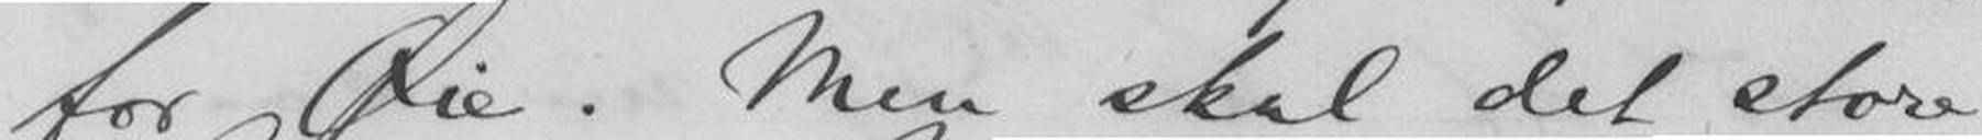for Øie. Men skal det store
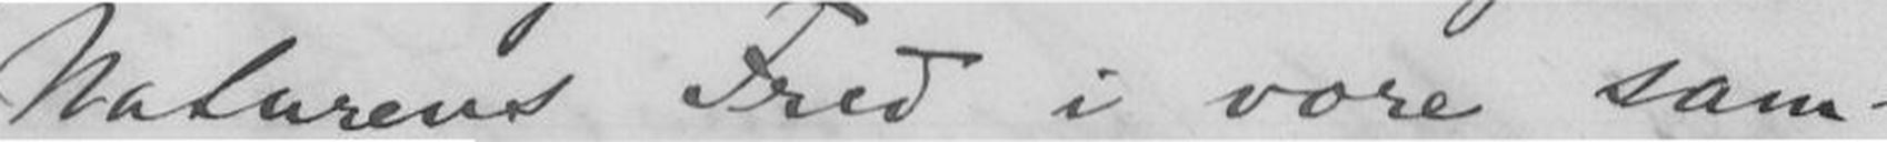Naturens Fred i vore sam-
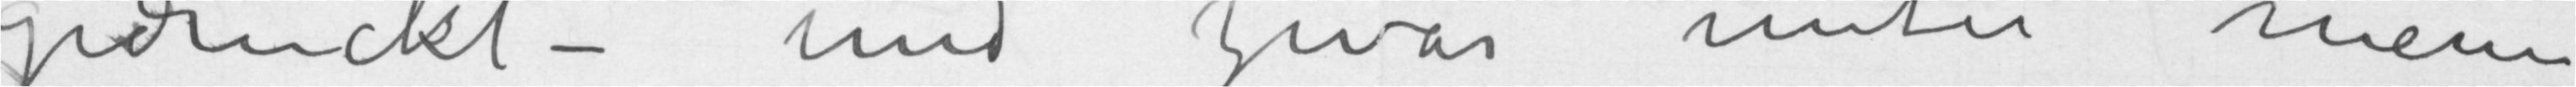gedruckt – und zwar unter meine
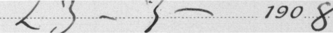23–3–1908
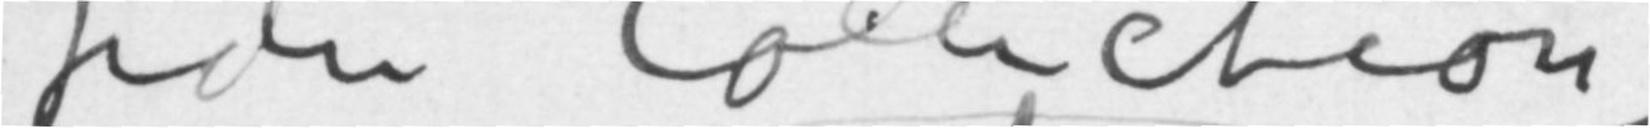jeden Collection
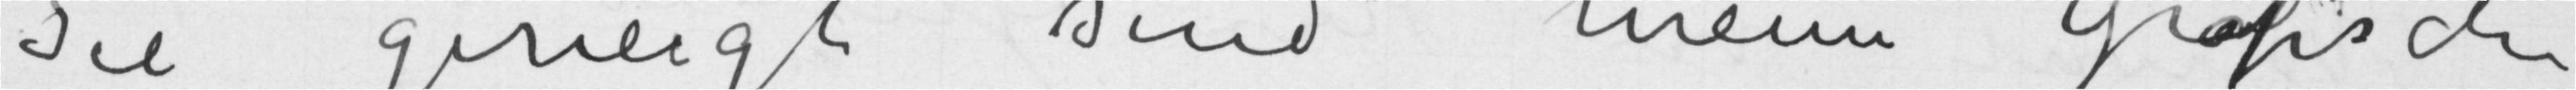Sie geneigt sind meine Grafischen
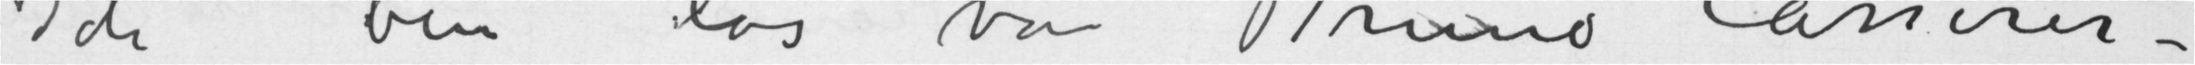Ich bin los von Bruno Cassirer –
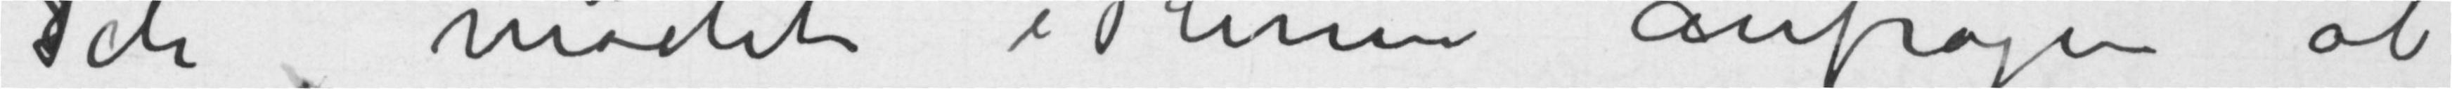Ich möchte Ihnen anfrage ob
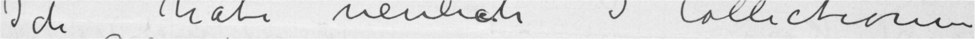Ich habe neulich 3 Collectionen
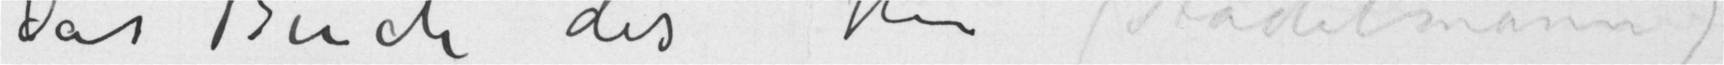Das Buch der Hrr (Stadelmann)
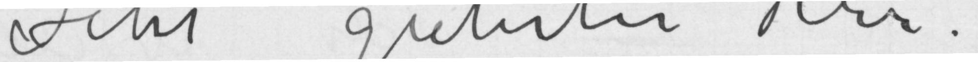Sehr geehrter Hrr!
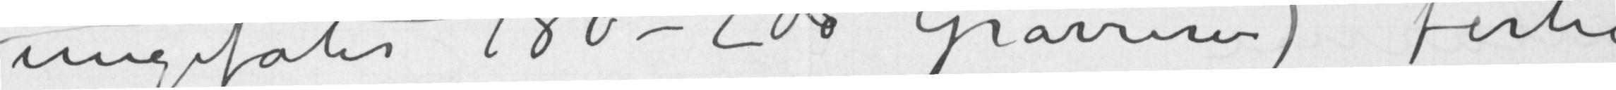ungefahr 180 – 200 Gravuren) fertig
